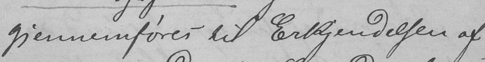gjennemføres til Erkjendelsen af
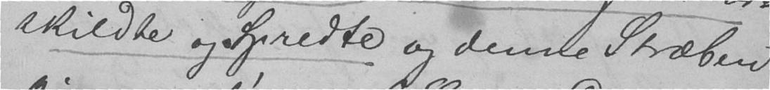skildte og spredte og denne Stræben
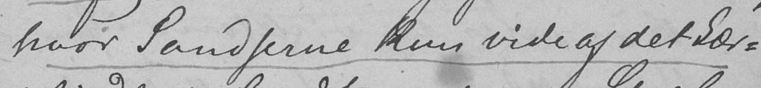hvor Sandserne kun vide af det sær-
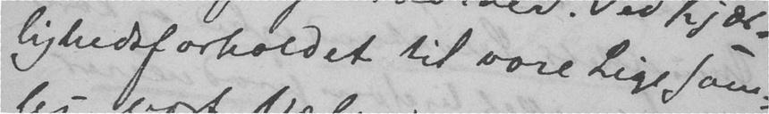lighedsforholdet til vore Lige sam-
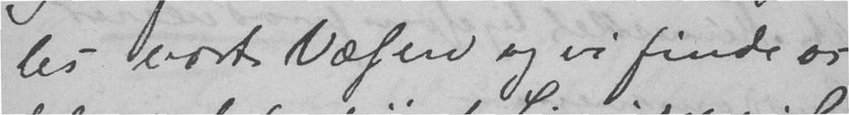les vort Væsen og vi finde os
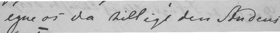egne os da tillige den Aandens
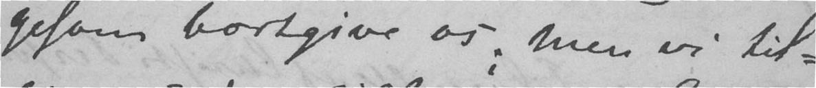gesom bortgive os; men vi til-
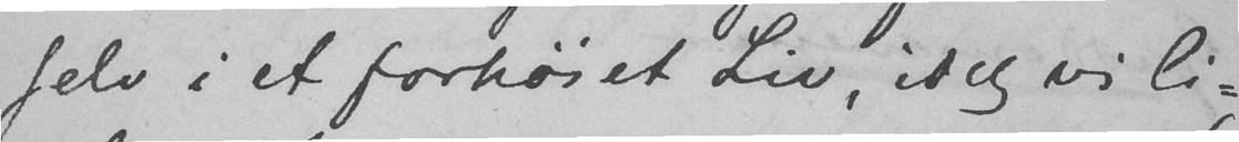selv i et forhøiet Liv, idet vi li-
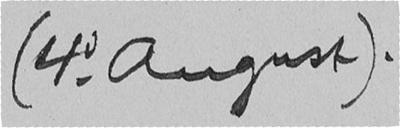(4d. August).
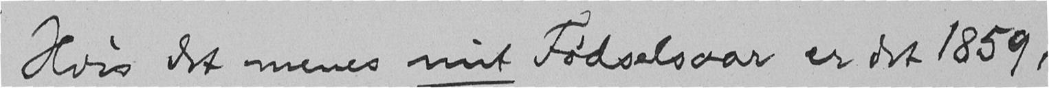Hvis det menes mit Fødselsaar er det 1859,
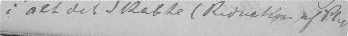i alt det Skabte (Reduction af Pligt)
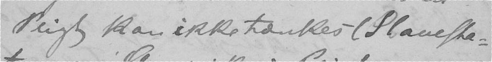Pligt kan ikke tænkes (Slavesta-
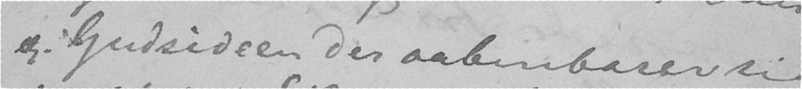gj. Gudsideen der aabenbarer sig
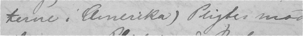terne i Amerika) Pligter mod
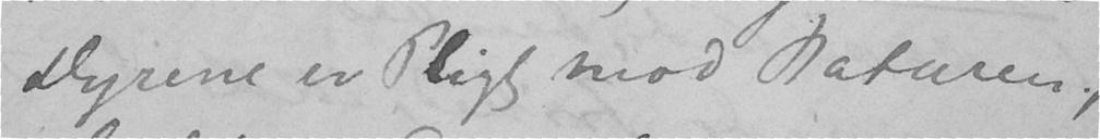Dyrene er Pligt mod Naturen,
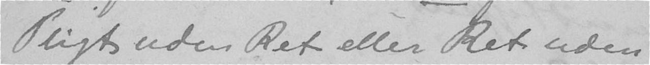Pligt uden Ret eller Ret uden
